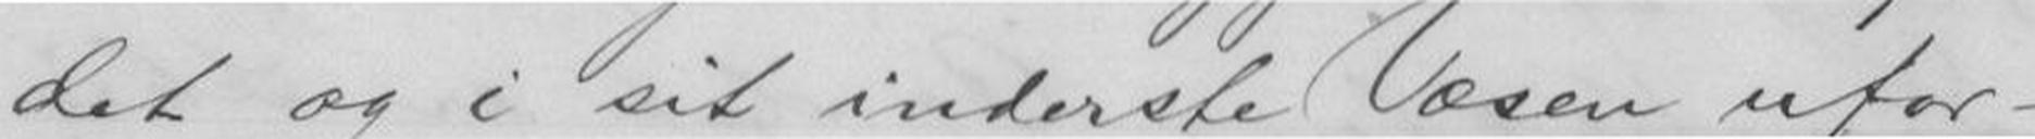det og i sit inderste Væsen ufor-
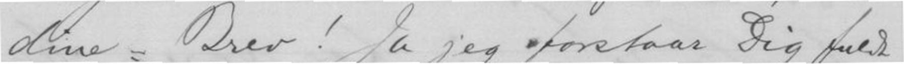dine=Brev! Ja jeg forstaar Dig fuldt
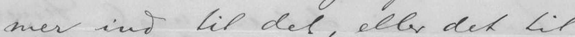mer ind til det, eller det til
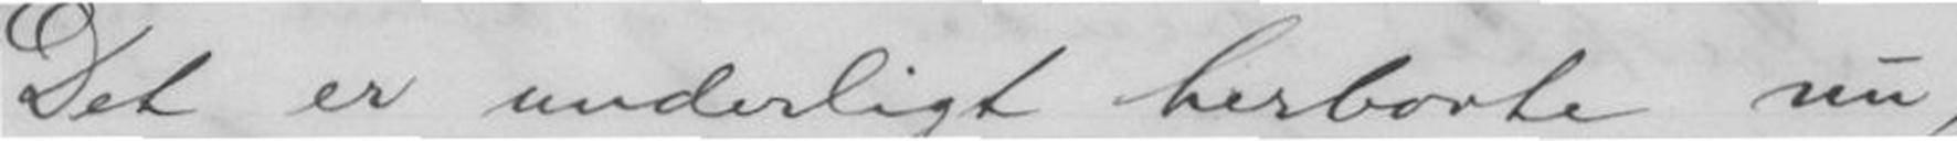Det er underligt herborte nu,
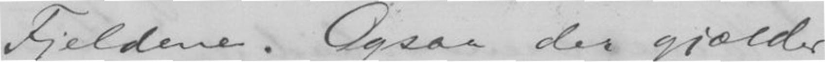Fjeldene. Ogsaa der gjælder
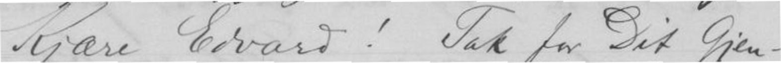Kjære Edvard! Tak for Dit Gjen-
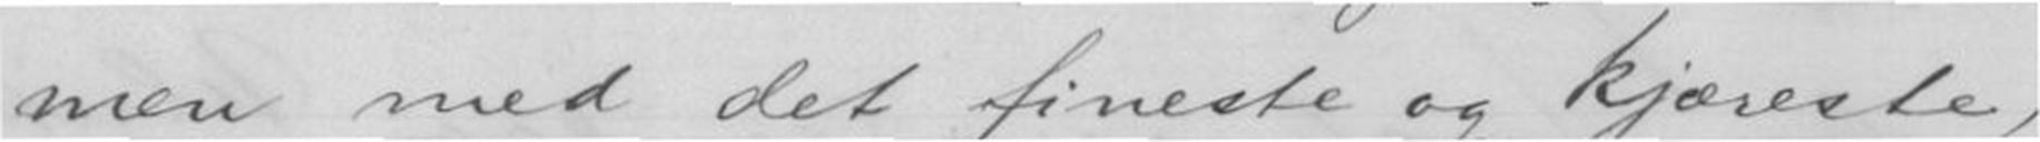men med det fineste og kjæreste,
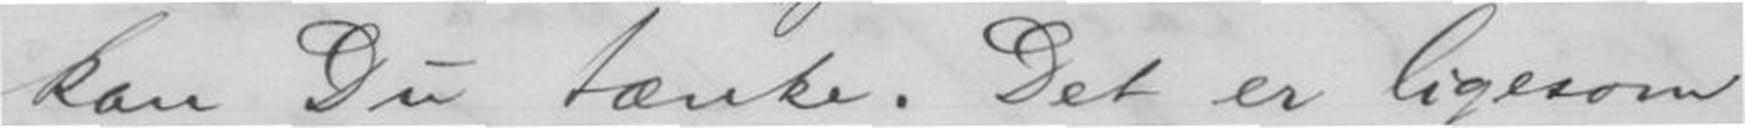kan Du tænke. Det er ligesom
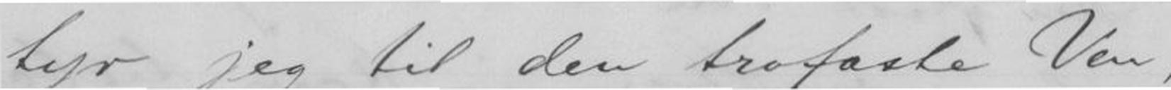tyr jeg til den trofaste Ven,
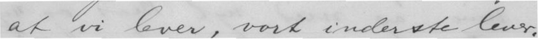at vi lever, vort inderste lever.
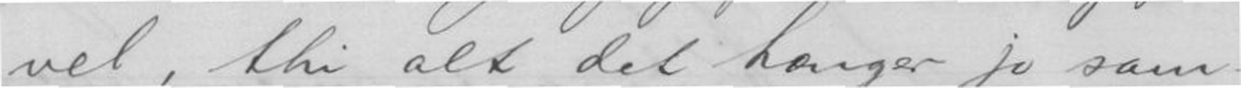vel, thi alt det hænger jo sam-
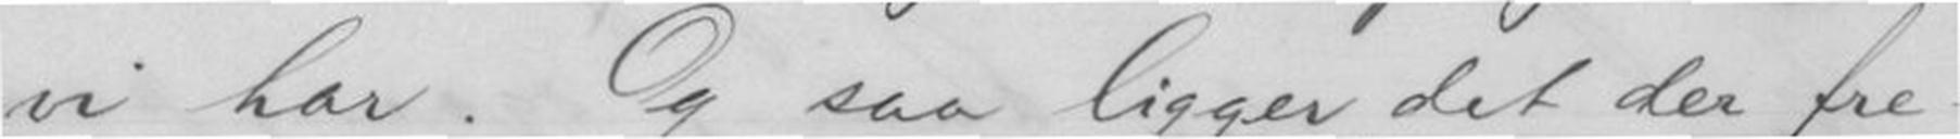vi har. og saa ligger det der fre-
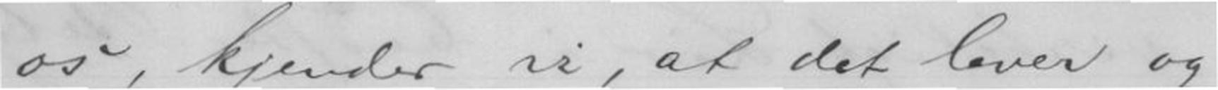os, kjender vi, at det lever og
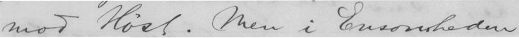mod Høst. Men i Ensomheden
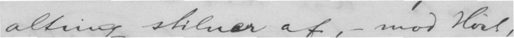alting stilner af, - mod Hvil,
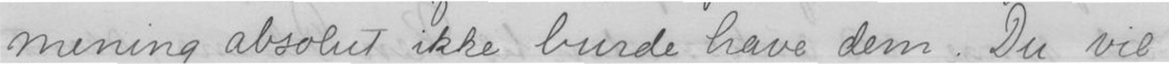mening absolut ikke burde have dem. Du vil
